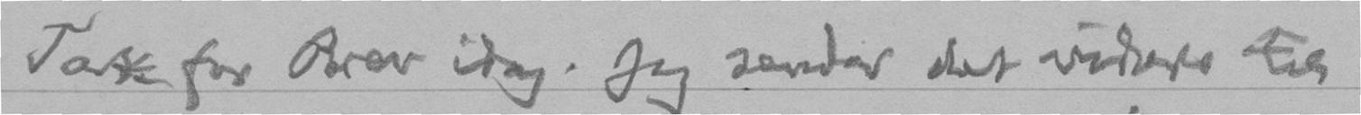Tak for Brev idag. Jeg sender det videre til
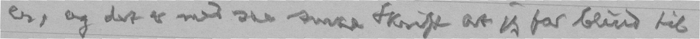er, og det er med saa smaa Skrift at jeg for blind til
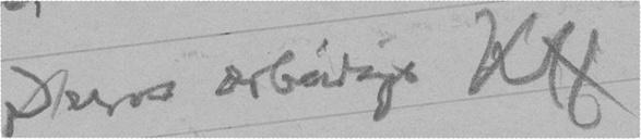Deres ærbødige KH
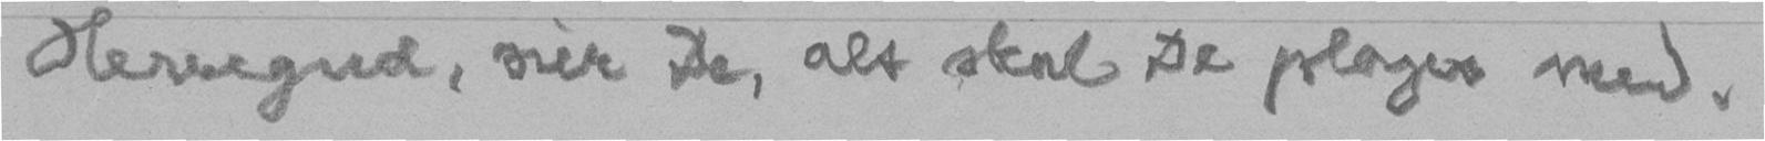Herregud, sier De, alt skal De plages med.
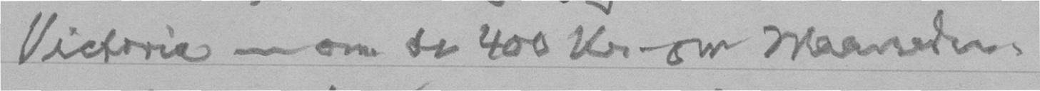Victoria - om de 400 Kr. om Maaneden.
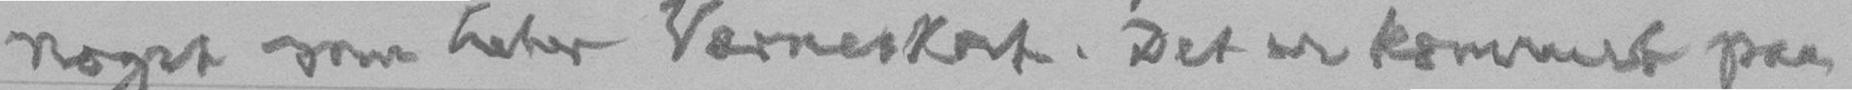noget som heter Værneskat. Det er kommet paa
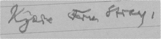Kjære Fru Stray,
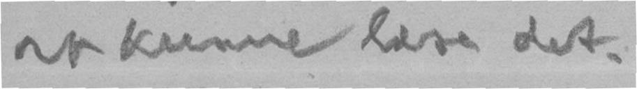at kunne læse det.
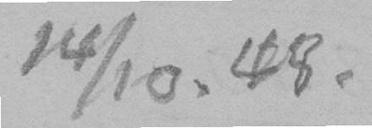14/10. 48.
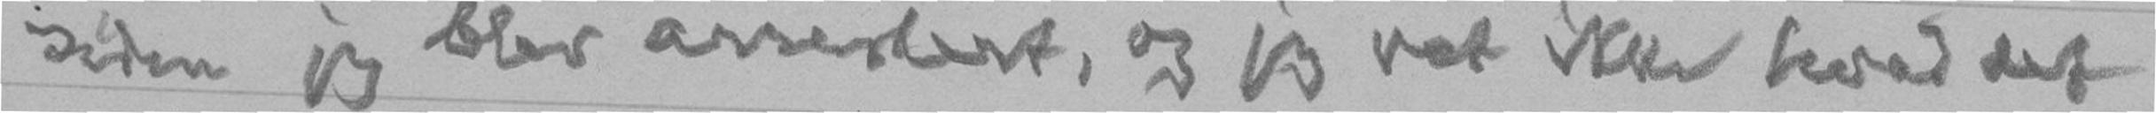siden jeg blev arrestert, og jeg vet ikke hvad det
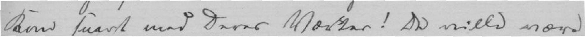Kom snart med Deres Værker! De ville være
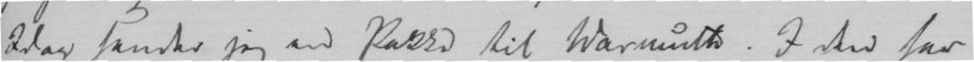Idag sender jeg en Pakke til Warmuth. I den har
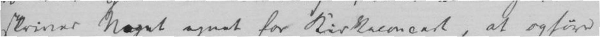skriver Noget egnet til Kirkeconcert, at opføre
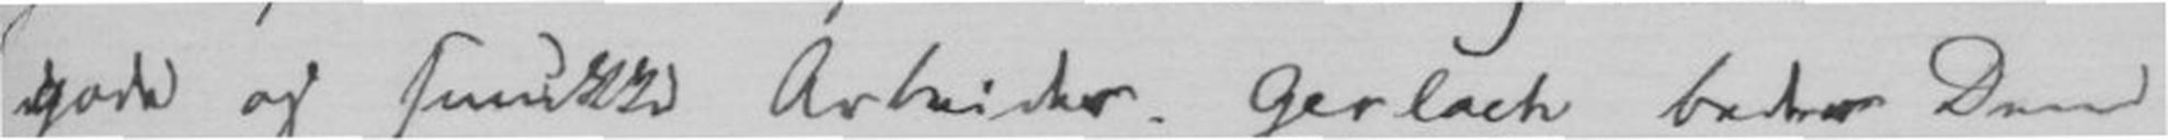gode og smukke Arbeider. Gerloch beder Dem
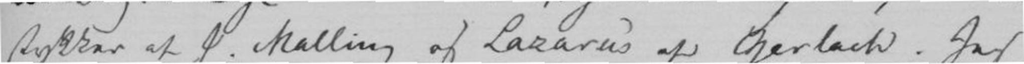stykker af J Malling og Lazarus af Gerloch. Jeg
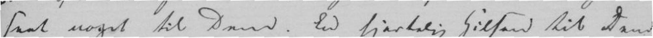seet noget til Dem. En hjertelig Hilsen til Dem
